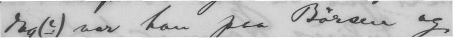dag(?) var han paa Børsen og
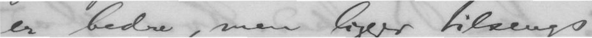er bedre, men ligger tilsengs
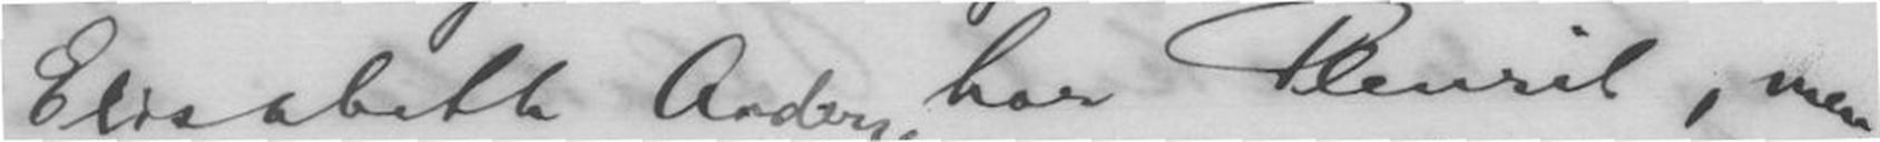Elisabeth Andersen har Pleurit, men
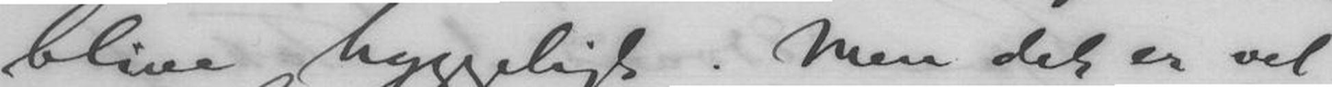blive hyggeligt. Men det er vel
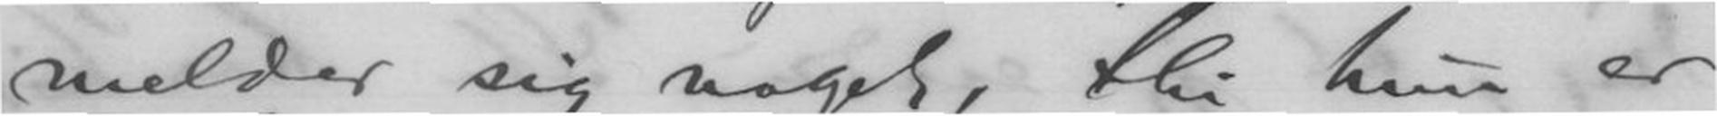melder sig noget, thi hun er
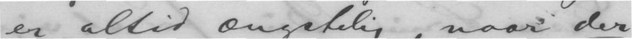er altid ængstelig, naar der
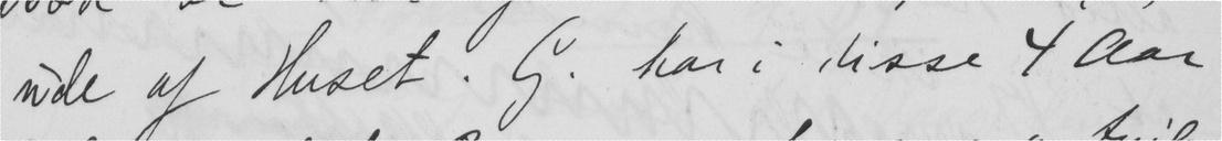ude af Huset. G. har i disse 4 Aar
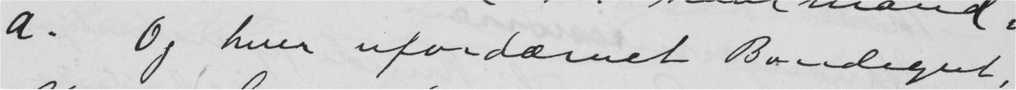a. Og hver ufordærnet Bondegut,
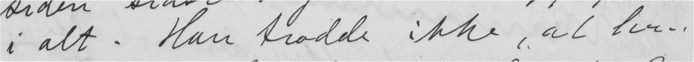i alt. Han trodde ikke at han
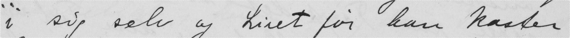i sig selv og Livet før han kaster
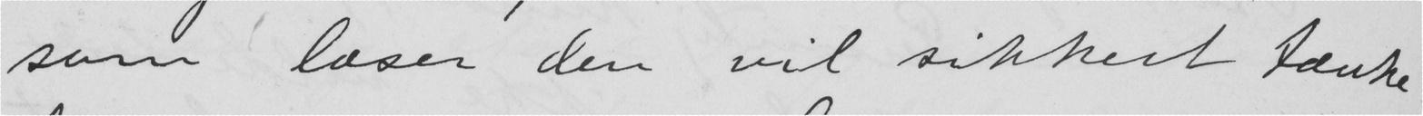som læser den vil sikkert tænke
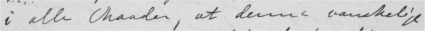i alle Maader, at denne vanskelige
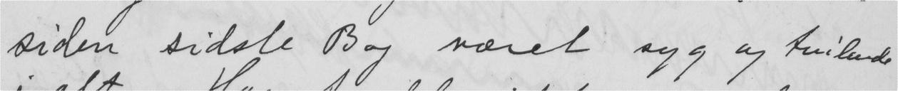siden sidste Bog været syg og tvilende
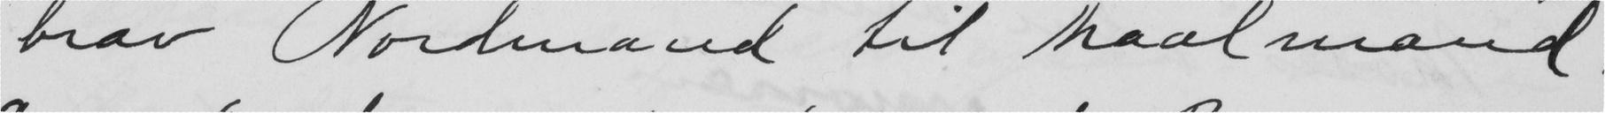brav Nordmand til Maalmand
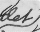det
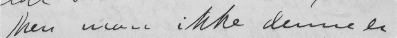Men mon ikke denne er
